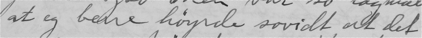at eg berre höyrde sovidt at det
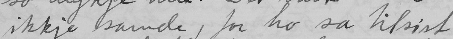ikkje samde, for ho sa tilsist
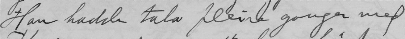Han hadde tala fleire gonger med
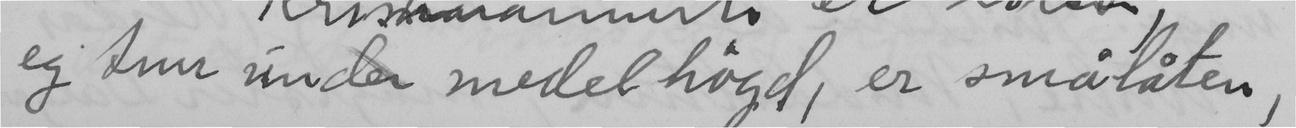eg trur under medel högd, er smålåten,
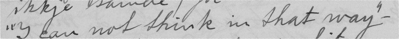"I can not think in that way" –
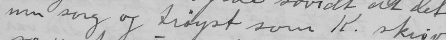um sorg og tröyst som K. skriv
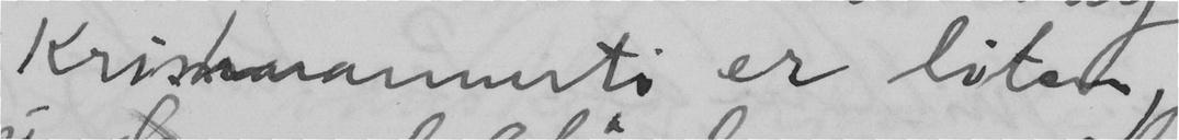Krishnamurti er liten,
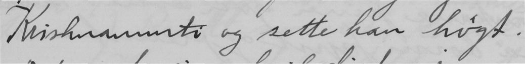Krishnamurti og sette han högt.
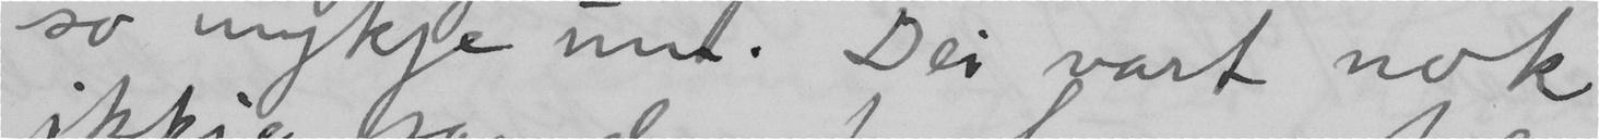so mykje um. Dei vart nok
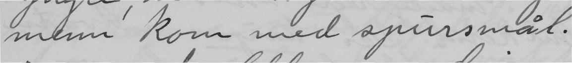menn kom med spursmål.
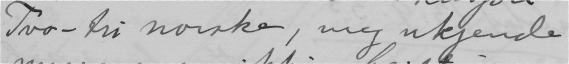Tvo-tri norske, meg ukjende
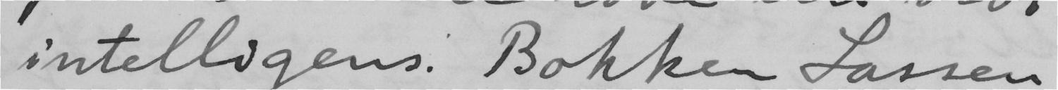intelligens. Bokken Lassen
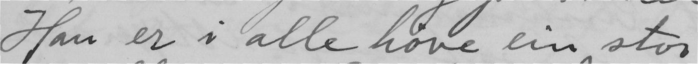Han er i alle höve ein stor
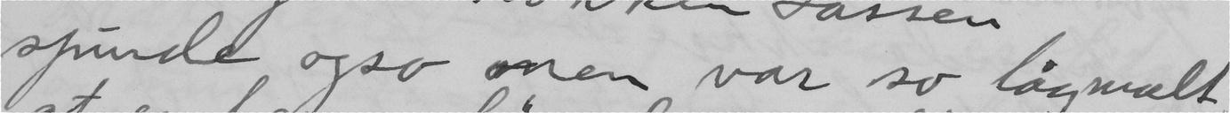spurde ogso men var so lågmalt
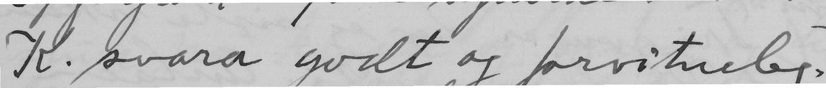K. svara godt og forvitneleg.
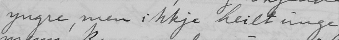yngre, men ikkje heilt unge
